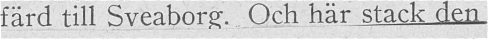färd till Sveaborg. Och här stack den
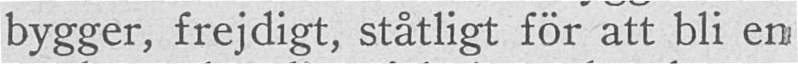bygger, frejdigt, stätligt för att bli en
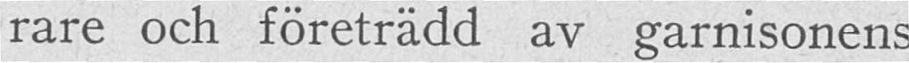rare och företrädd av garnisonens
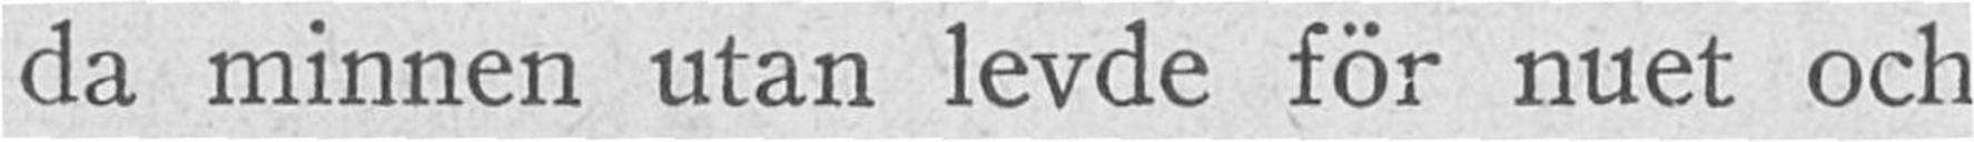da minnen utan levde för nuet och
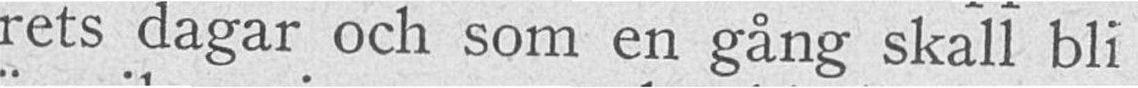rets dagar och som en gang skall bli
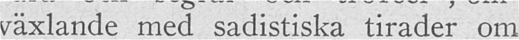växlande med sadistiska tirader om
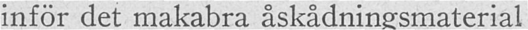infor det makabra åskådningsmaterial
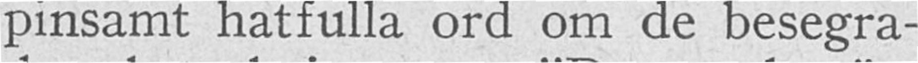pinsamt hatfulla ord om de besegra-
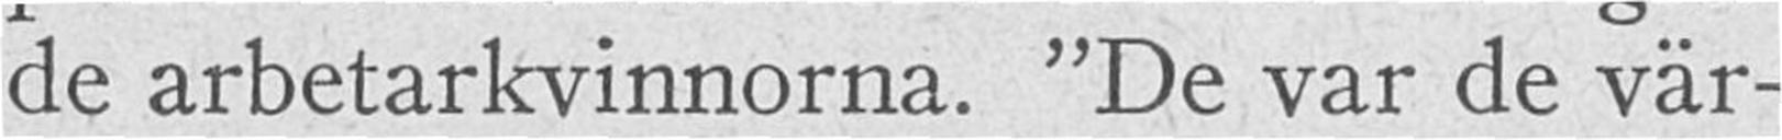de arbetarkvinnorna. "De var de vär-
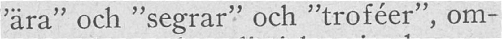"ära och "segrar" och "troféer", om-
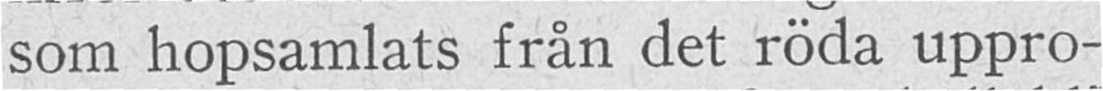som hopsamlats från det roda uppro-
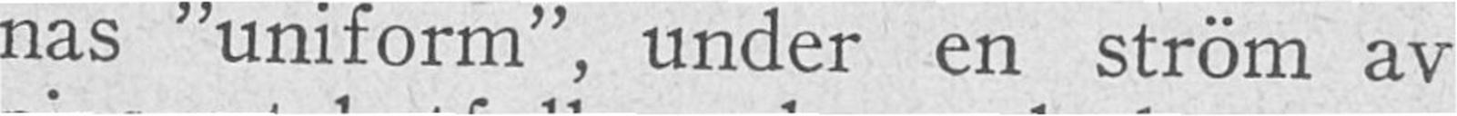nas "uniform" under en strôm av
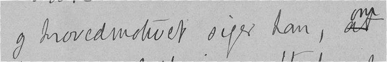og hovedmotivet siger han, at
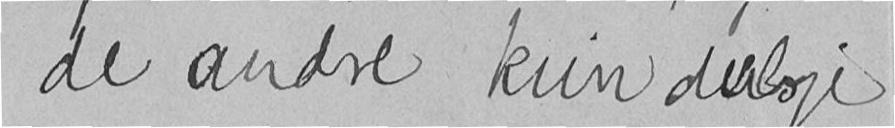de andre kvindelige
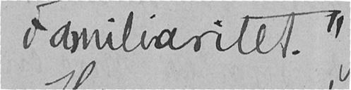Familiaritet."
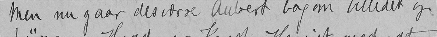Men nu gaar desværre Aubert bagom billedet og
